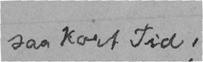saa kort Tid,
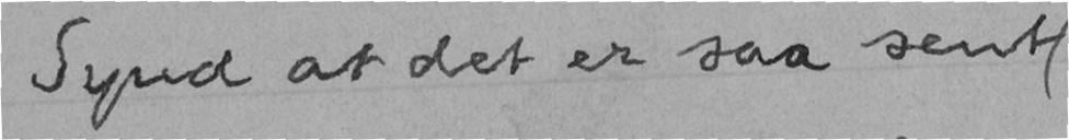Synd at det er saa sent
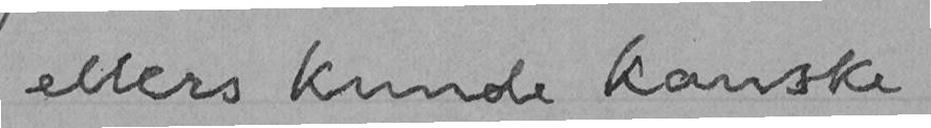ellers kunde kanske
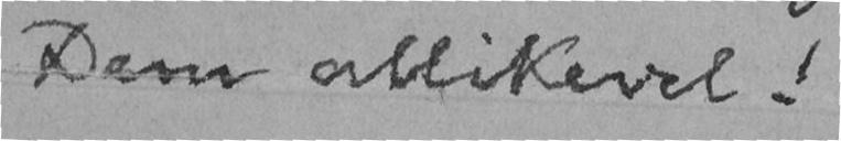Dem allikevel!
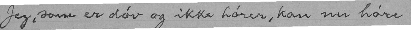Jeg, som er døv og ikke hører, kan nu høre
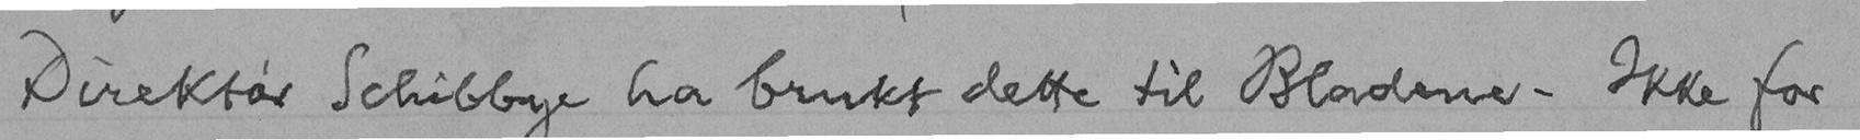Direktør Schibbye ha brukt dette til Bladene. Ikke for
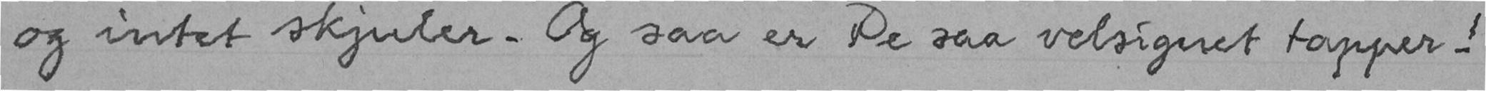og intet skjuler. Og saa er De saa velsignet tapper!
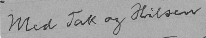Med Tak og Hilsen
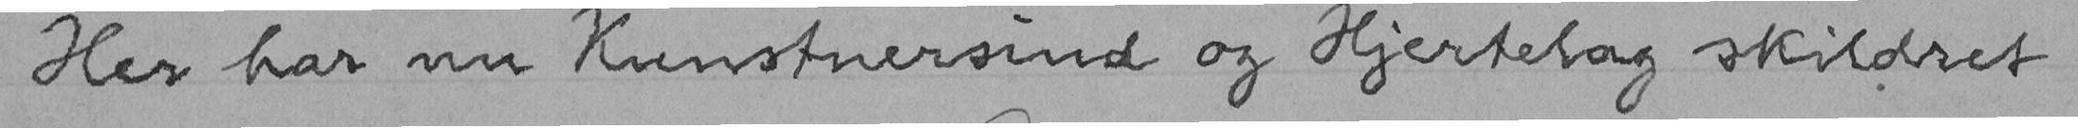Her har nu Kunstnersind og Hjertelag skildret
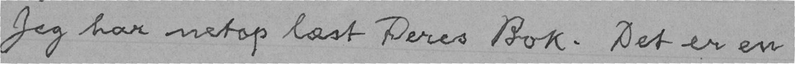Jeg har netop læst Deres Bok. Det er en
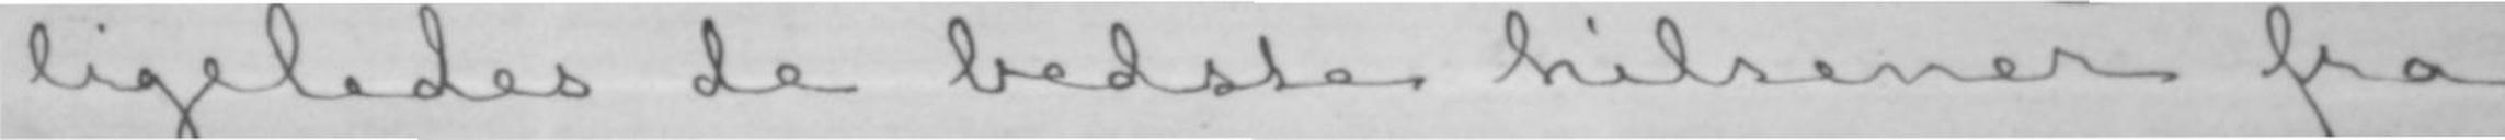ligeledes de bedste hilsener fra
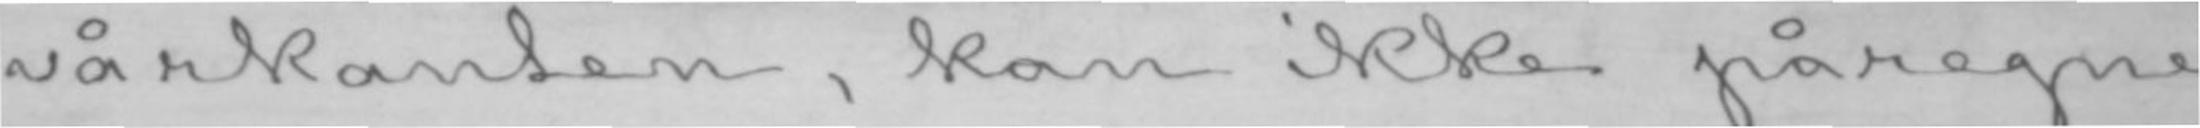vårkanten, kan ikke påregne
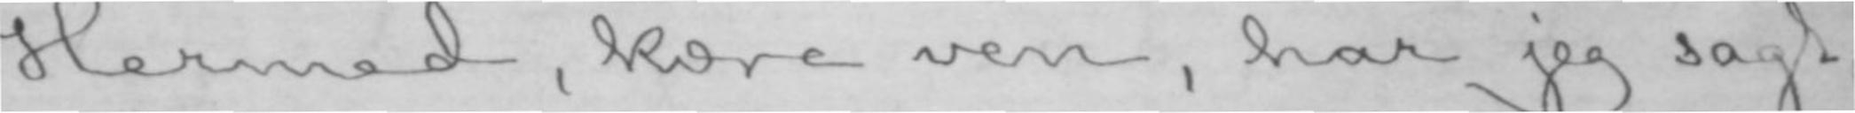Hermed, kære ven, har jeg sagt,
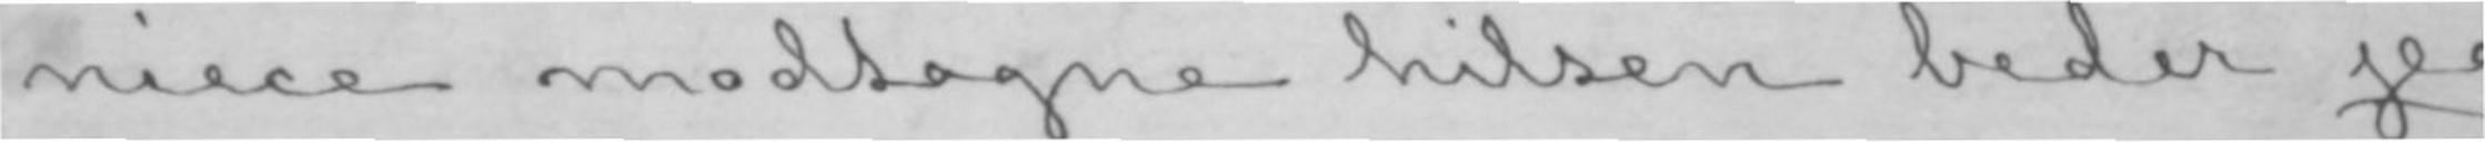niece modtagne hilsen beder jeg
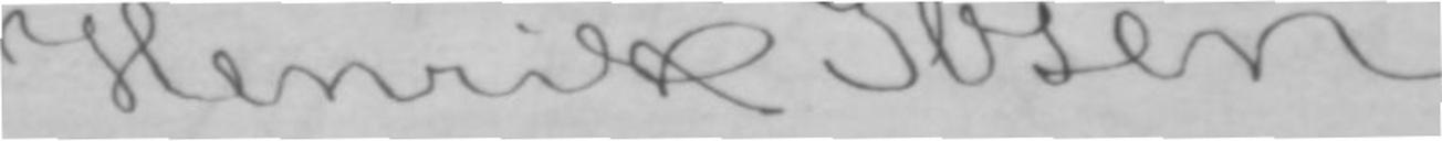Henrik Ibsen.
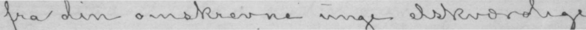fra din omskrevne unge elskværdige
